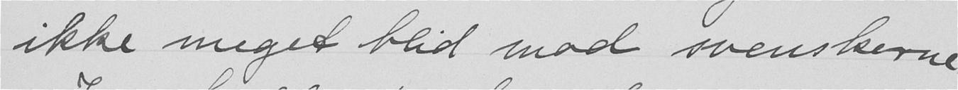ikke meget blid mod svenskerne.
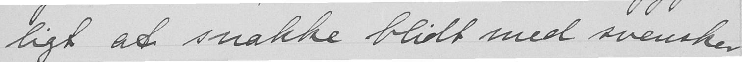ligt at snakke blidt med svensker
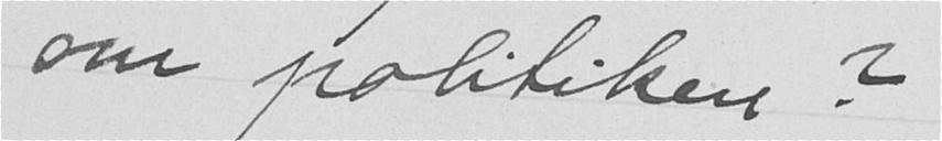om politiken?
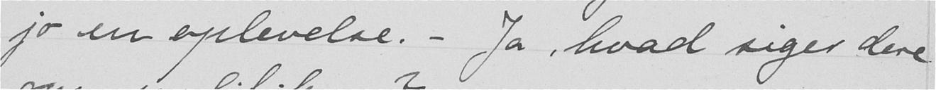jo en oplevelse. - Ja, hvad siger dere
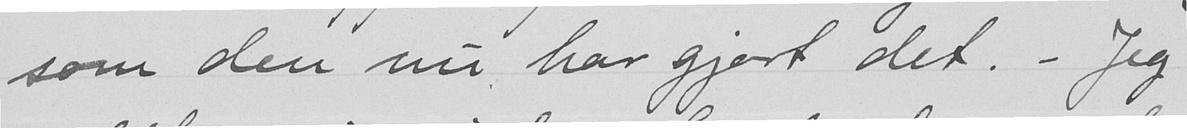som den nu har gjort det. - Jeg
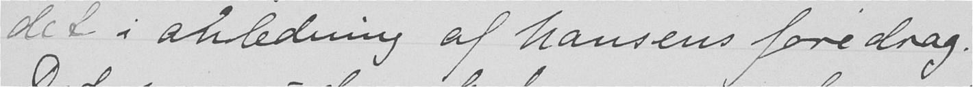det i anledning af Nansens foredrag.
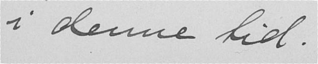i denne tid.
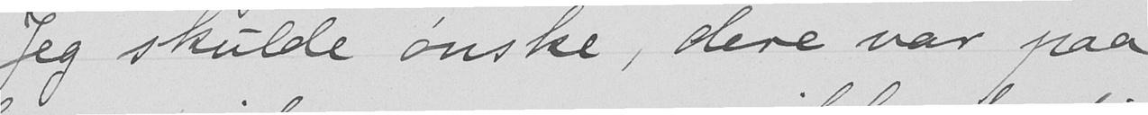Jeg skulde ønske, dere var paa
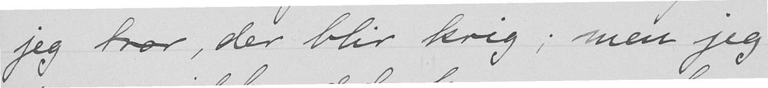jeg tror, der blir krig; men jeg
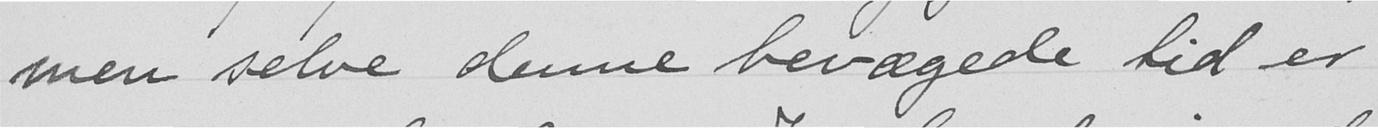men selve denne bevægede tid er
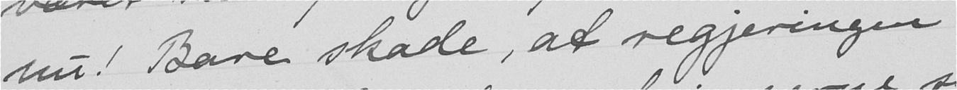nu! Bare skade, at regjeringen
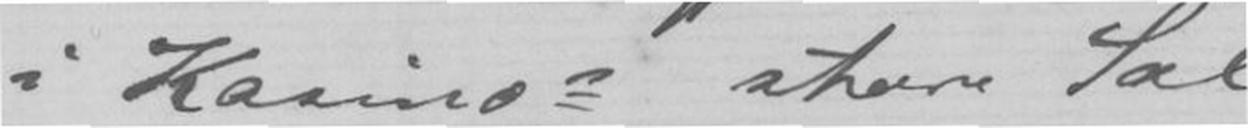i Kasinos store Sal
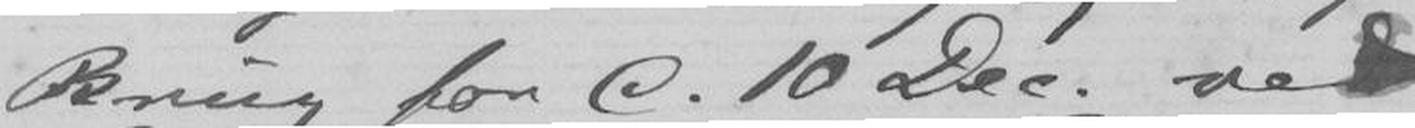Brug for d. 10 Dec. ved
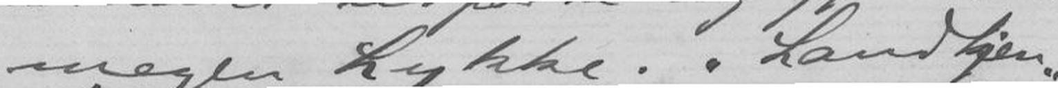megen Lykke. Landkjen-
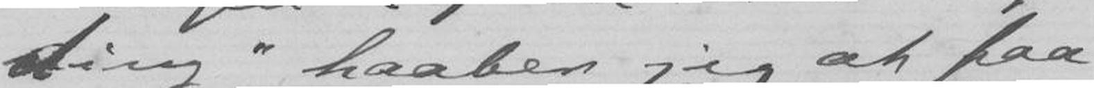ding haaber jeg at faa
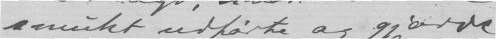smukt udførte og gjorde
